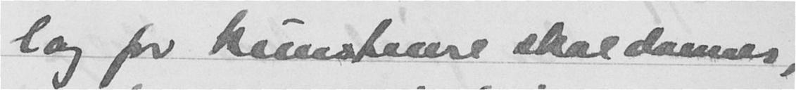lag for kunsterne skal dannes,
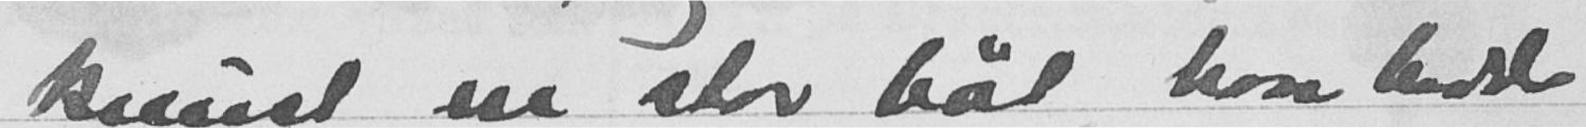knust en stor båt, han hadde
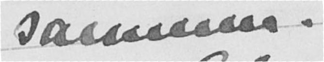sammen.
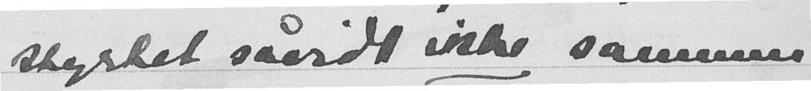styrtet såvidt ikke sammen
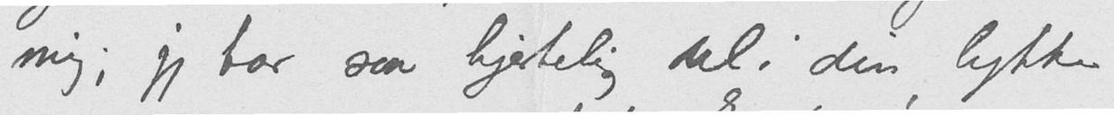mig; jeg tar saa hjertelig del i din lykke
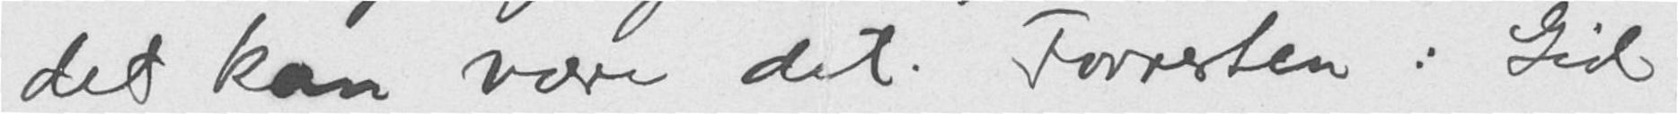det kan være det. Forresten: Gid
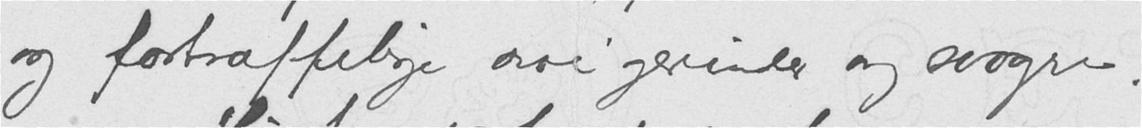og fortræffelige svigerinder og svogre.
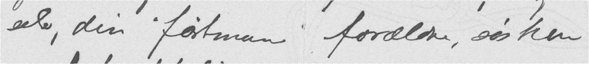selv, din "fästman", forældre, søsken
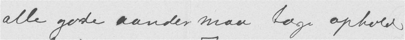alle gode aander maa tage ophold
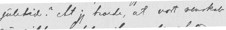juletid? At jeg troede, at vort venskab
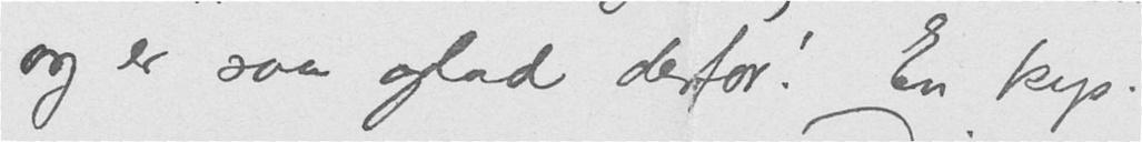og er saa glad derfor! En kys!
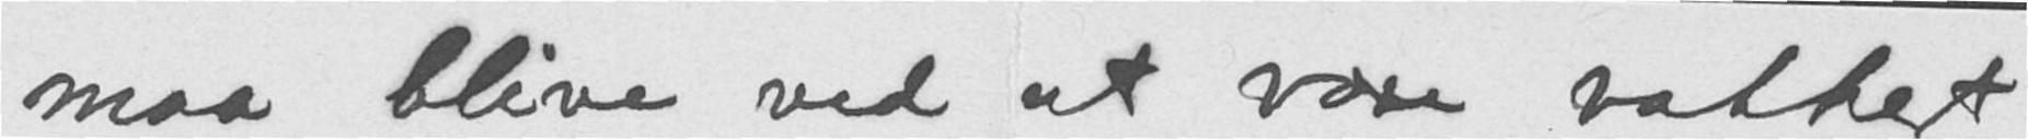maa blive ved at være vakkert
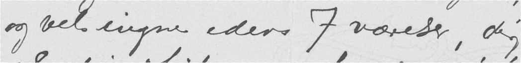og velsigne eders 7 værelser, dig
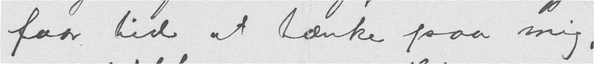faar tid til at tænke paa mig,
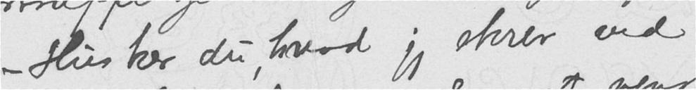- Husker du, hvad jeg skrev ved
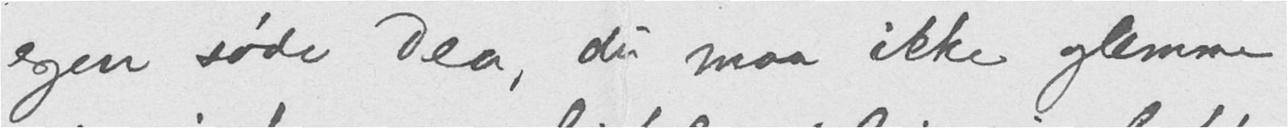egen søde Dea, du maa ikke glemme
